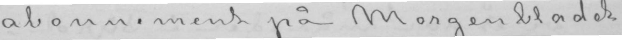abonnement på «Morgenbladet»,
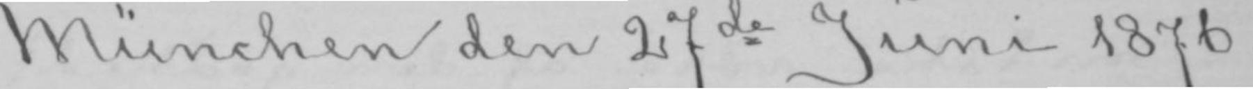München den 27de Juni 1876.
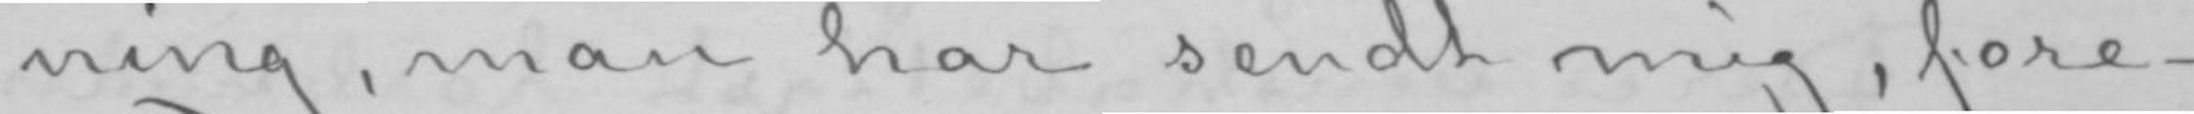ning, man har sendt mig, fore-
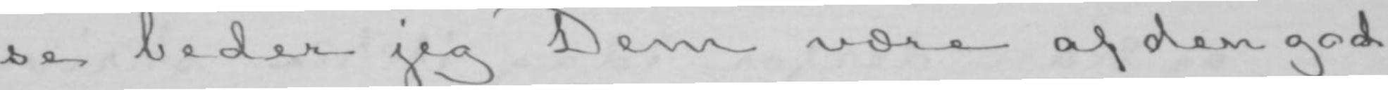se beder jeg Dem være af den god-
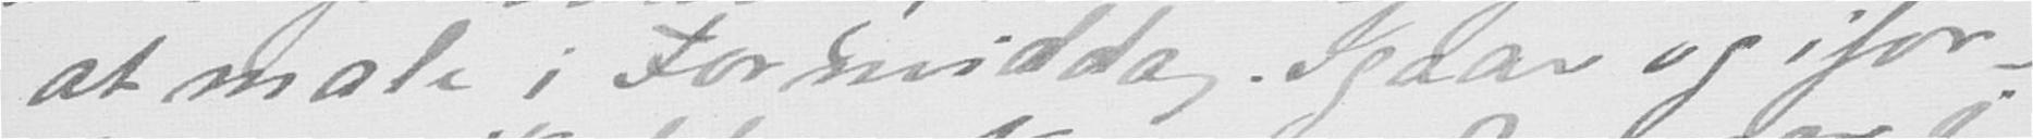at male i Formiddag. Igaar og ifor-
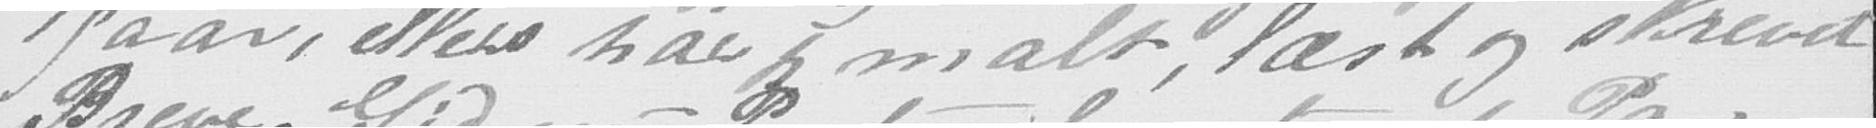igaar, ellers har jeg malt, læst og skrevet
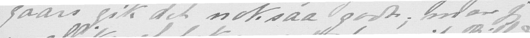gaars gik det noksaa godt, men jeg
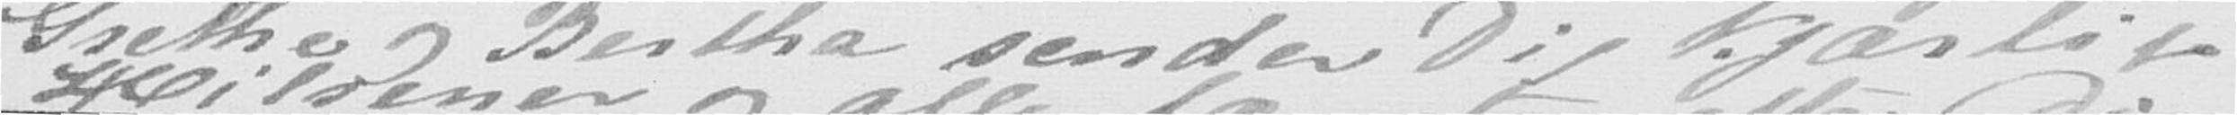Grethe og Bertha sender dig Kjærlige
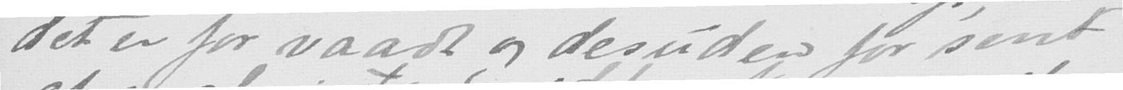det er for vaadt og desuden for sent,
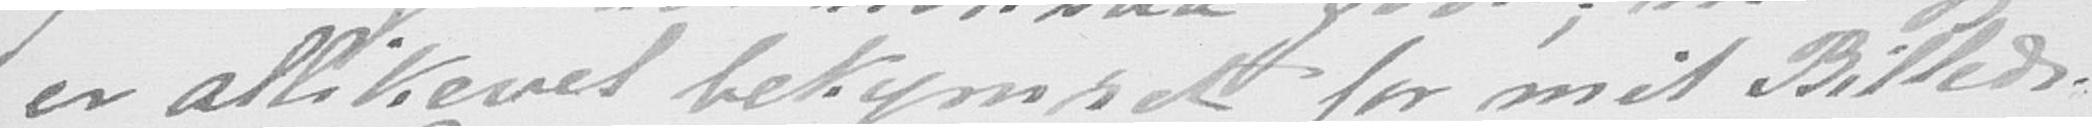er allikevel bekymret for mit Billed.
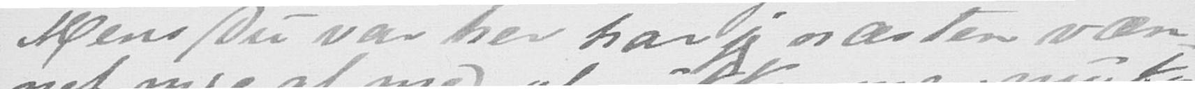Mens Du var her har jeg næsten væn-
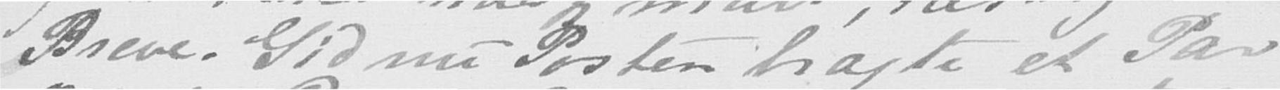Breve. Gid nu Posten bragte et Par
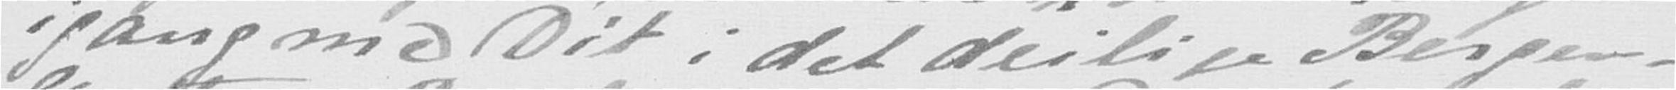igang med Dit i det deilige Bergen.
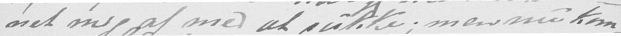net mig af med at sukke; men nu kom-
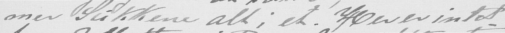men Sukkene alt i et. Her er intet
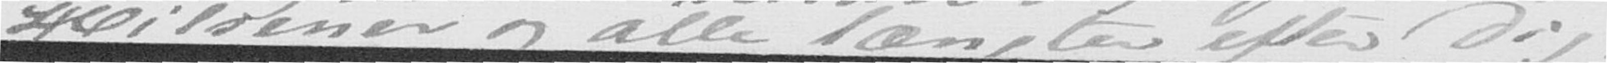Hilsener og alle længter efter Dig.
